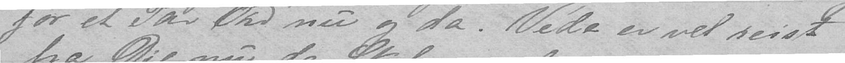for et Par Ord nu og da. Veda er vel reist
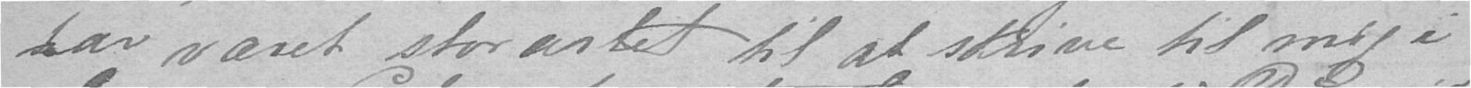har været storartet til at skrive til mig i
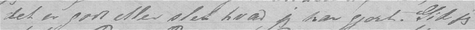det er godt eller slet hvad jeg har gjort. Gid jeg
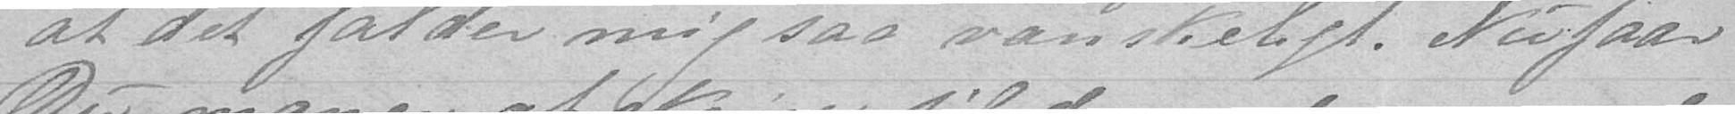at det falder mig saa vanskeligt. Nu faar
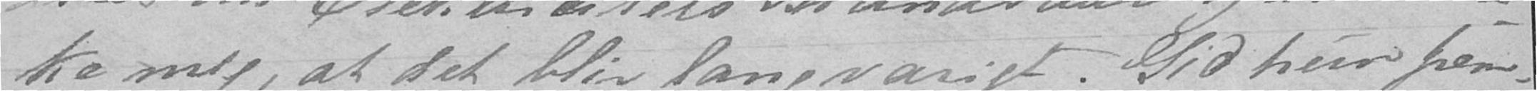ke mig, at det blir langvarigt. Gid hun frem-
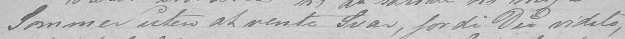Sommer uten at vente Svar, fordi Du vidste,
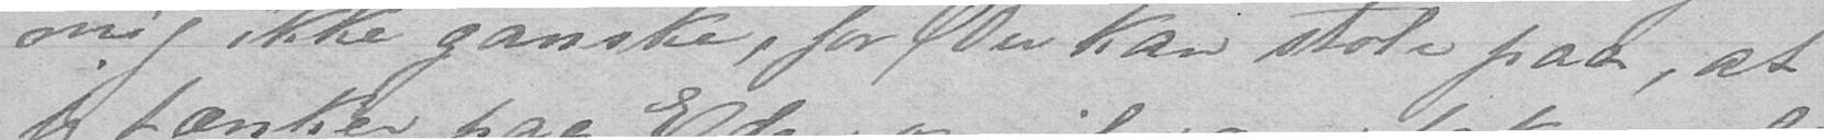mig ikke ganske, for Du kan stole paa, at
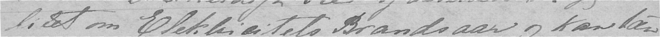lidet om Elekricitets Brandsaar og kan tæn-
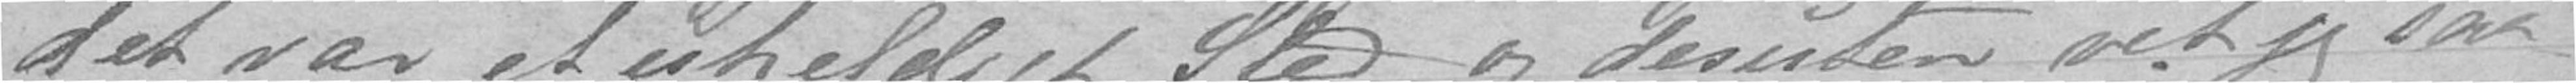det var et uheldigt Sted, og desuten vet jeg saa
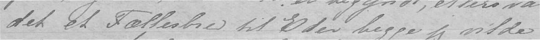det et Fællesbrev til Eder begge jeg vilde
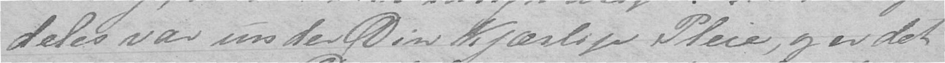deles var under Din Kjærlige Pleie, og er det
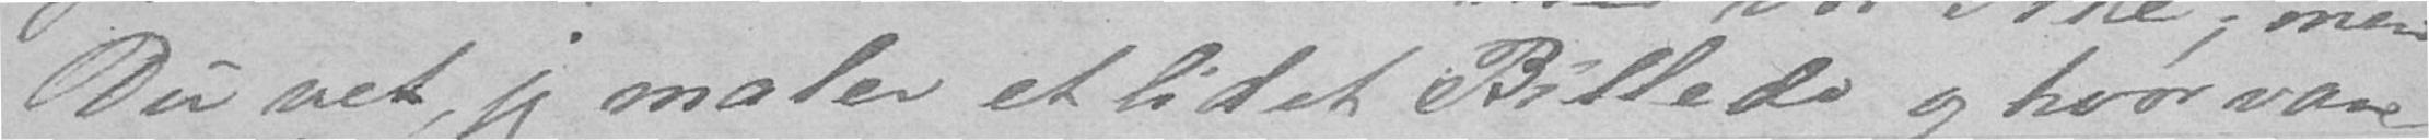Du vet, jeg maler et lidet Billede og hvor van-
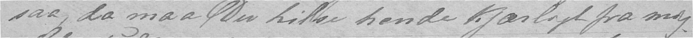saa, da maa Du hilse hende Kjærligt fra mig.
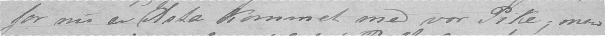for nu er Asta kommet med vor Pike; men
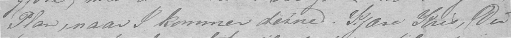Plan, naar I kommer derned. Kjære Kris, Du
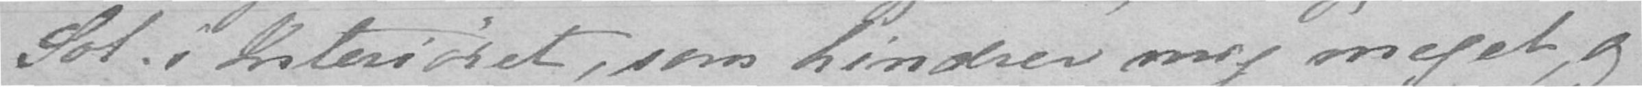Sol i Interiøret, som hindrer mig meget, og
